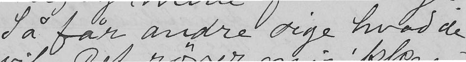Så får andre sige hvad de
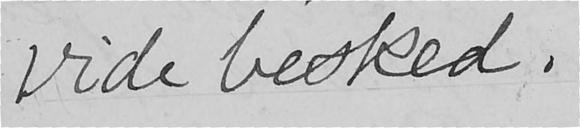vide besked.
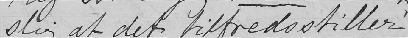slig at det tilfredsstiller
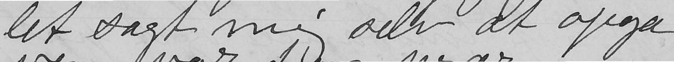let sagt mig selv at opga
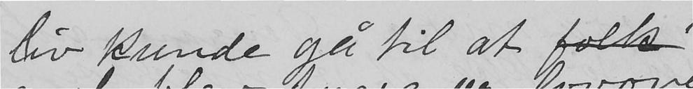liv kunde gå til at folk
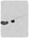-
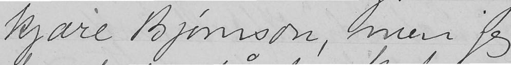kjære Bjørnson, men jeg
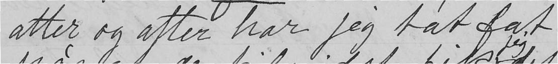atter og atter har jeg tat fat
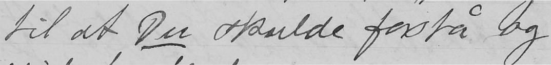til at Du skulde forstå og
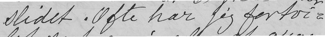slidet. Ofte har jeg fortvi-
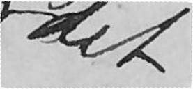det
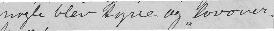nogle blev tyve og lovover-
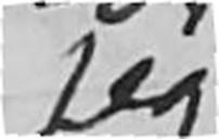jeg
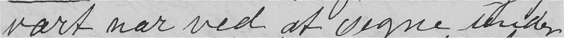vært nær ved at segne under
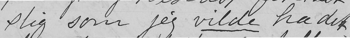slig som jeg vilde ha det,
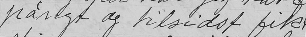pånyt og tilsidst fik
